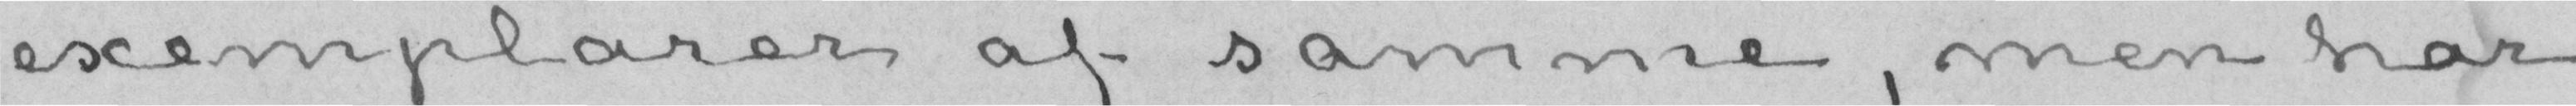exemplarer af samme, men har
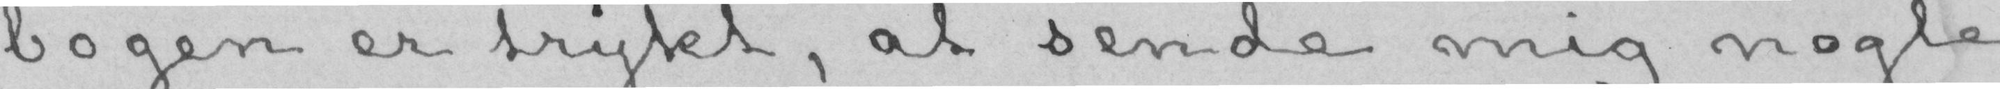bogen er trykt, at sende mig nogle
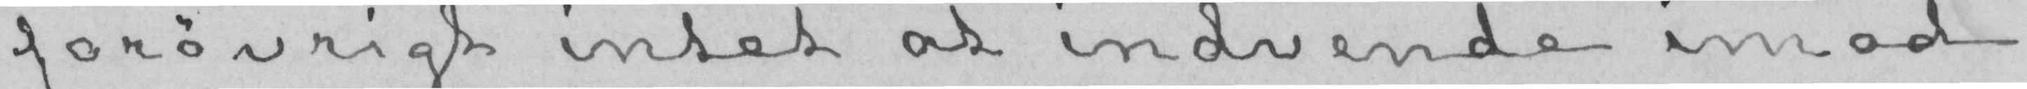forøvrigt intet at indvende imod,
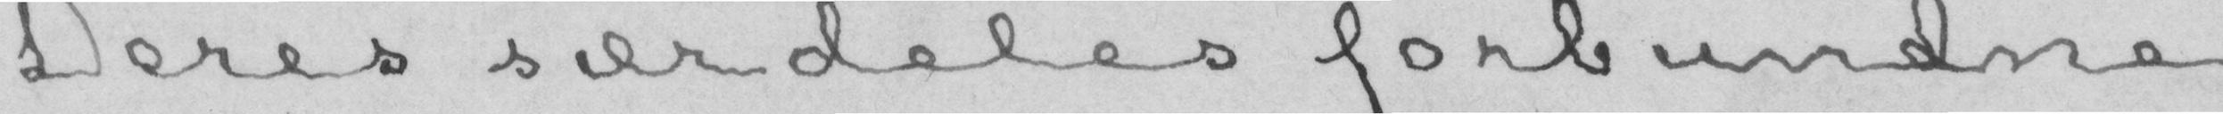Deres særdeles forbundne
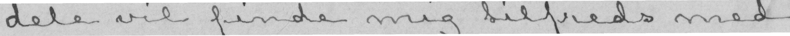dele vil finde mig tilfreds med
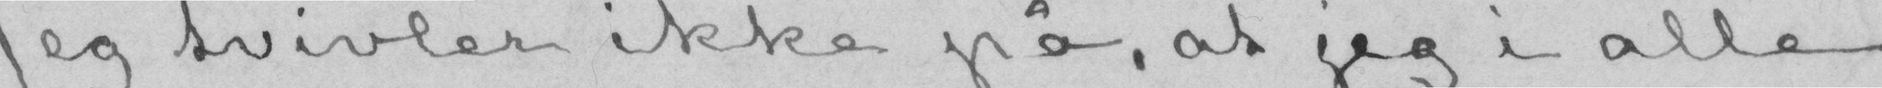Jeg tvivler ikke på, at jeg i alle
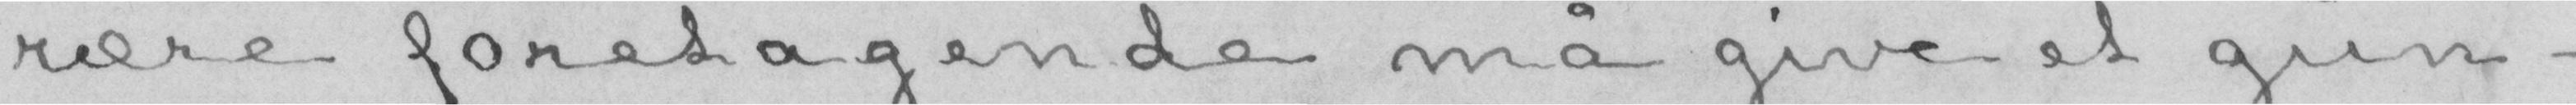rære foretagende må give et gun-
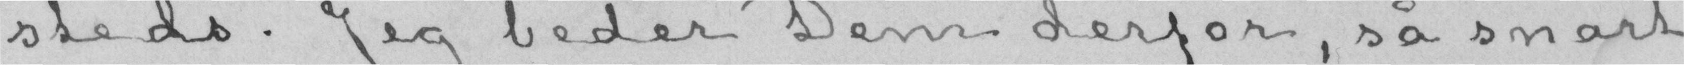steds. Jeg beder Dem derfor, så snart
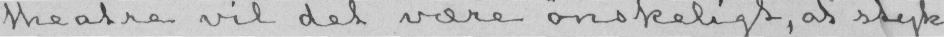theatre vil det være ønskeligt, at styk
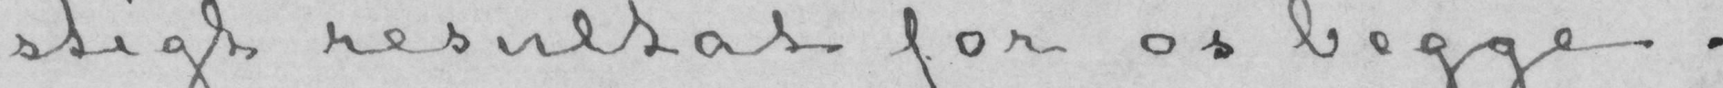stigt resultat for os begge.
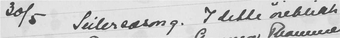20/5 Seilersesong. I dette øieblikk
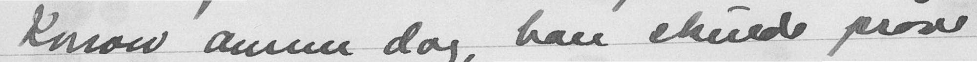Konow annen dag, han skulde prøve
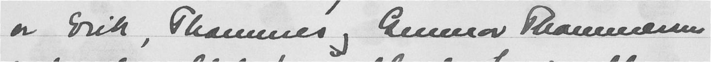er Erik, Thommes og Gunnar Thommessen
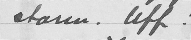storm. Uff!
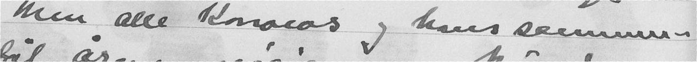Men alle Konowos og hans sammen-
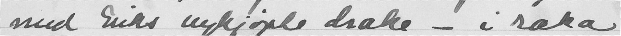med Eriks nykjøpte drake - i raka
